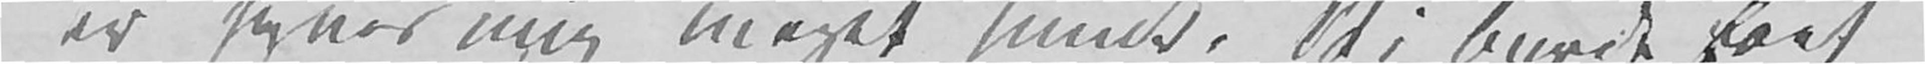er synes mig meget smuk. Vi burde fået
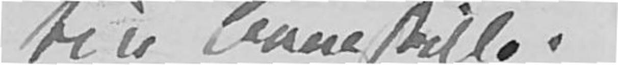tie bomstille.
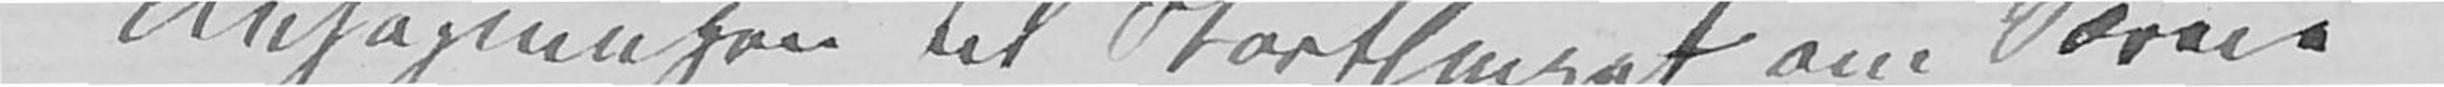Ansøgningen til Storthinget om Særeie
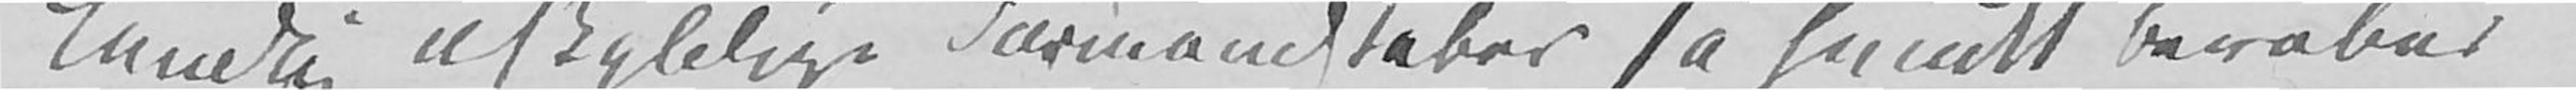landlig uskyldige Formandskaber så smukt beråber
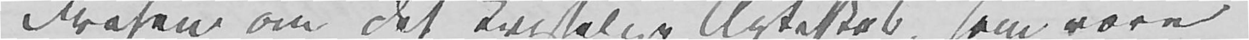Frasen om «det Kristelige Ægteskab», som vore
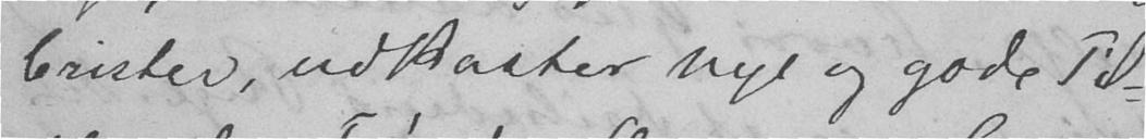brister, udkaster nye og gode Til-
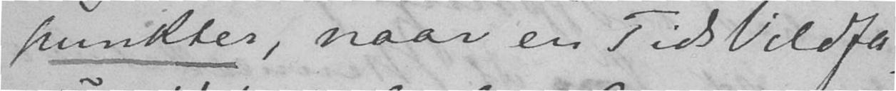punkter, naar en Tidsvildfa-
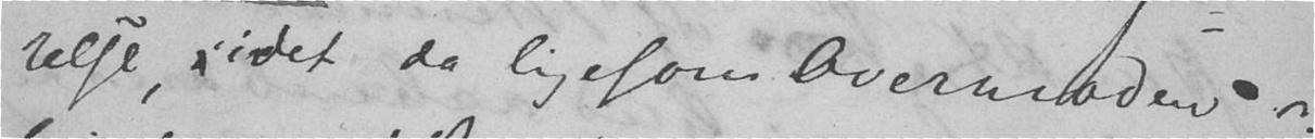relse, idet da ligesom Overmoden
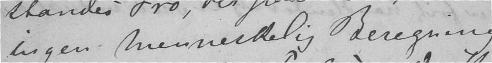ingen menneskelig Beregning
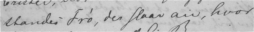standes Frø, der slaar an, hvor
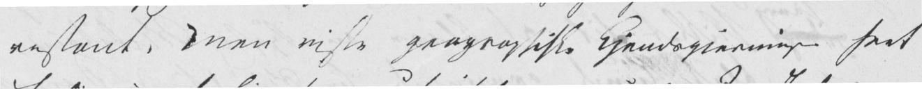ressant, men visse geographiske Kjendsgjerninger seet
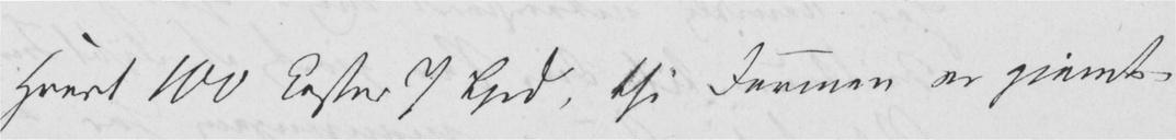Hvert 100 koster 7 Spd, thi Formen er gjemt.
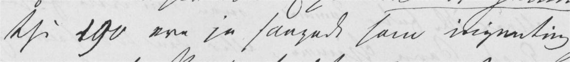thi 190 ere jo saagodt som ingenting
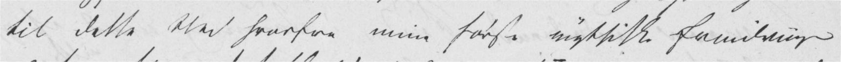til dette Sted hvorfra mine første mythiske Erindringer
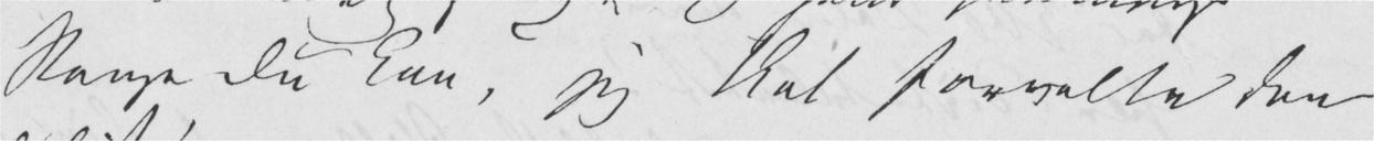Penge Du kan, jeg skal forvalte dem
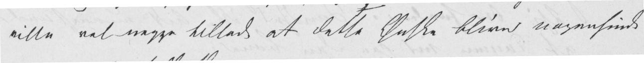ville vel neppe tillade at dette Ønske bliver nogensinde
